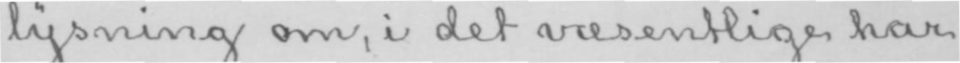lysning om, i det væsentlige har
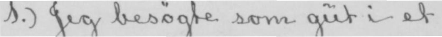1.) Jeg besøgte som gut i et
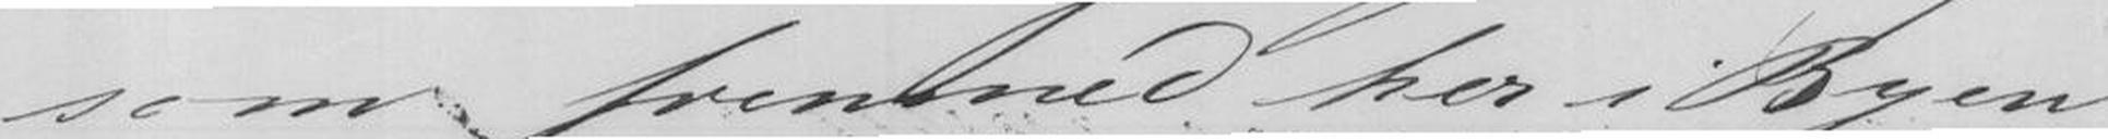som fremmed her i Byen
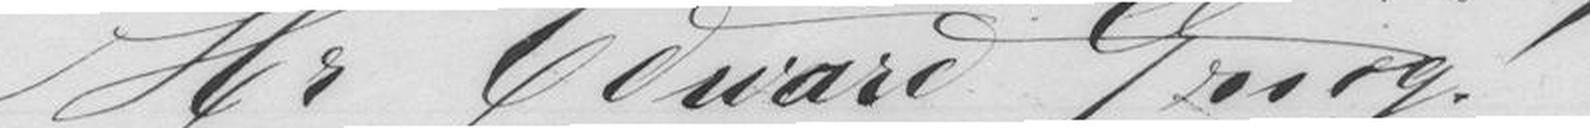Hr Edward Grieg!
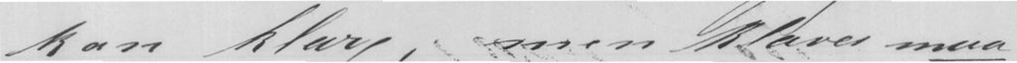kan klare; men klares maa
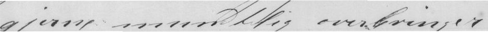gjerne mundtlig overbringer
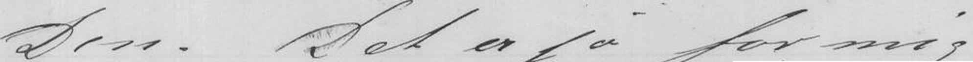Den. Det er jo for mig
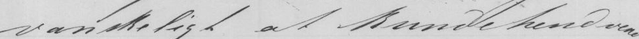vanskeligt at kunde hendvende
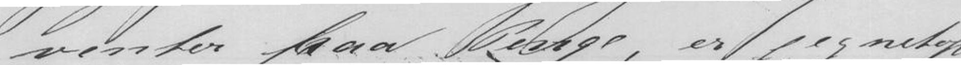venter paa Penge, er jeg netop
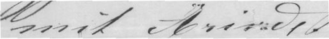mit Ærinde.
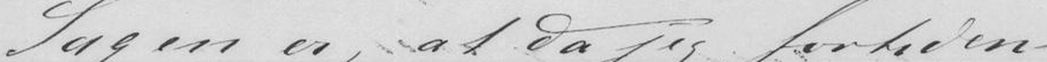Sagen er, at da jeg fortiden
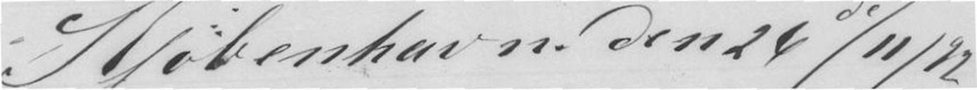Kjøbenhavn den 24de/11/92
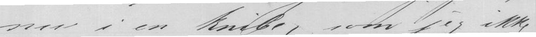nu i en knibe, som jeg ikke
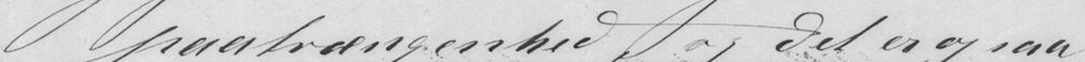paatrængenhed, og det er ogsaa
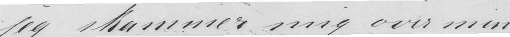Jeg skammer mig over min
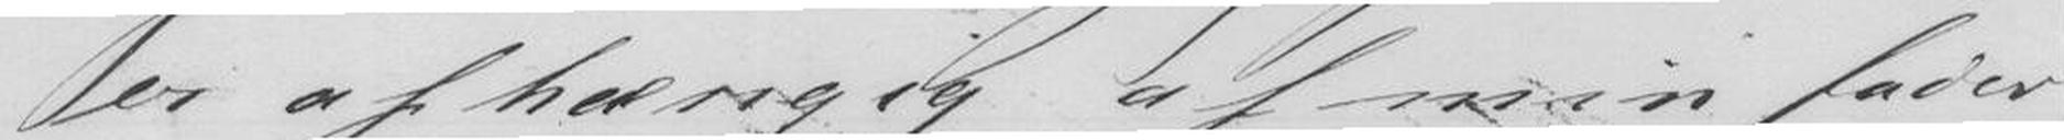er afhægig af min fader
